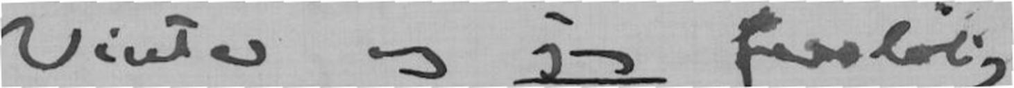Vinter og jeg foreløbig
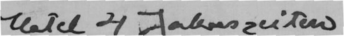Hotel 4 Jakreszuiten
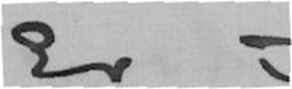Er I
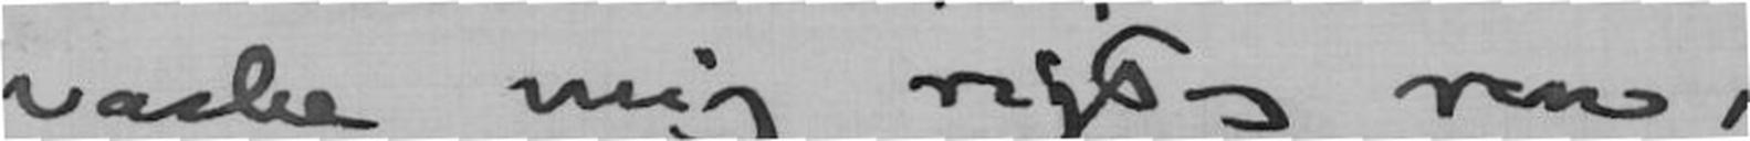vaske mig rigtig ren,
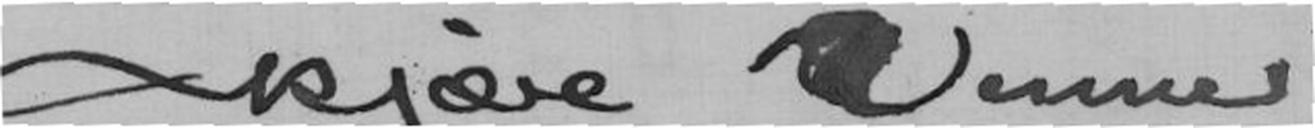Kjære Venner
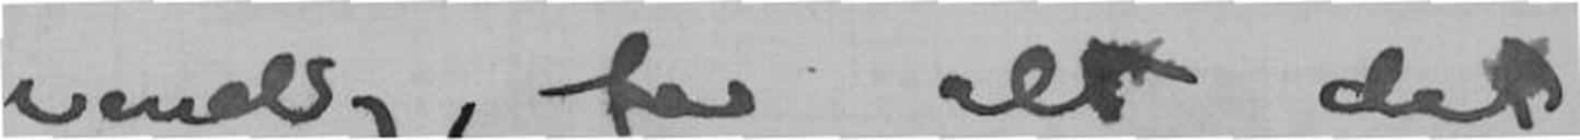vendig, for alt det
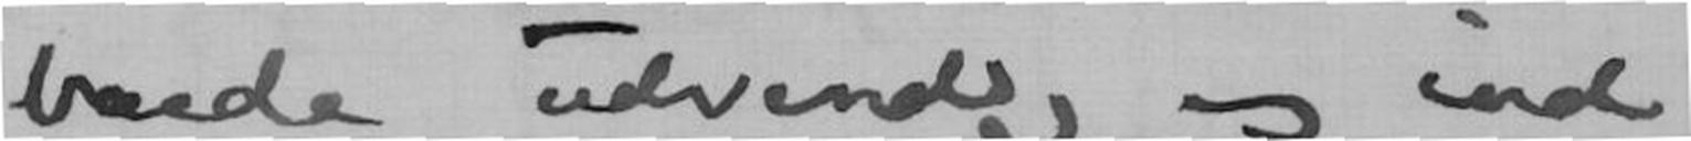baade udvendig og ind-
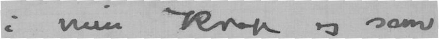i min Krop og som
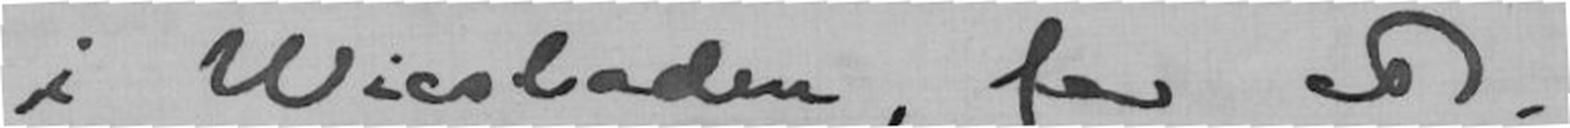i Wiesbaden, for at
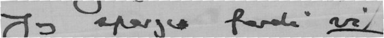Jeg spørger fordi vi
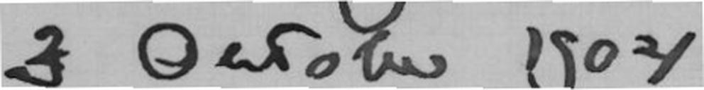3 October 1904
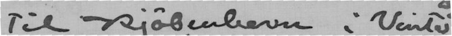til Kjøbenhavn i Vinter
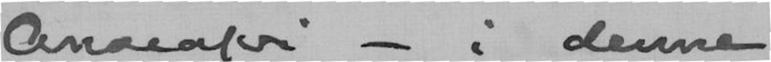Anacaferi - i denne
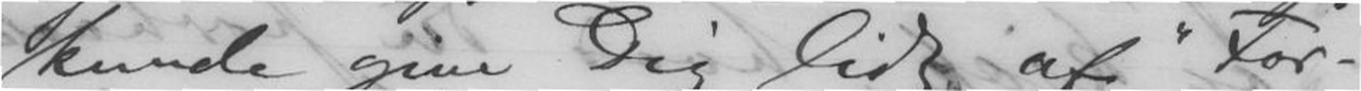kunde give Dig lidt af "For-
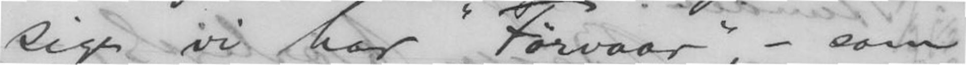sige vi har "Førvaar", - som
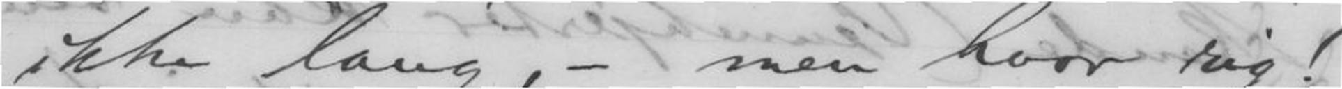ikke lang, - men hvor rig!
